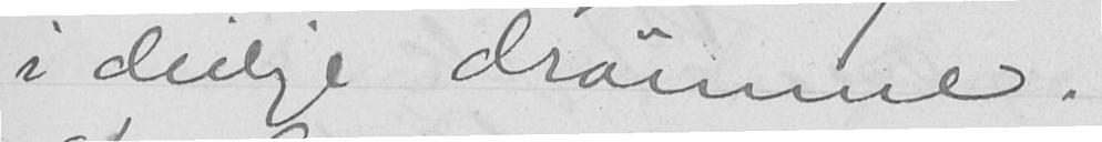i deilige drømme.
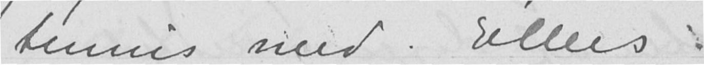tennis med. Ellers
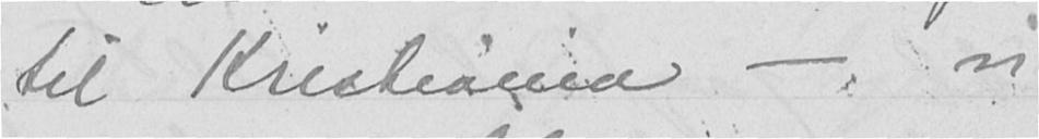til Kristiania - vi
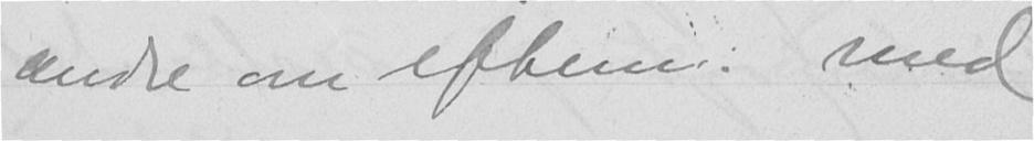andre om efterm. med
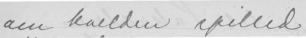om kvelden spilled
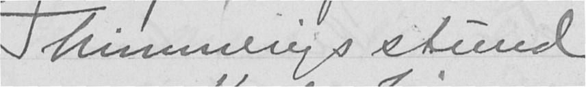himmerigsstund
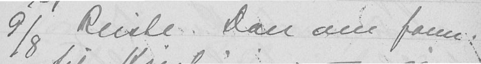9/8 Reiste Don om form.
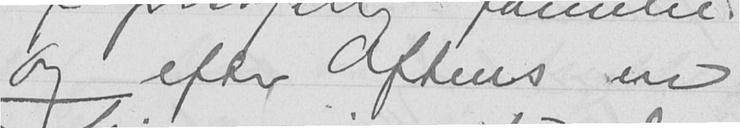Og efter aftens en
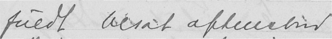fuldt besat aftensbord
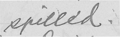spilled.
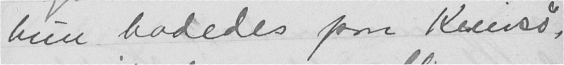hun badedes paa Knivsø,
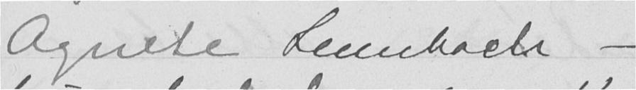Agnete Leuerbach -
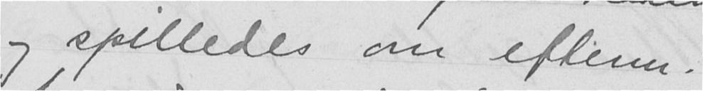og spilledes om efterm.
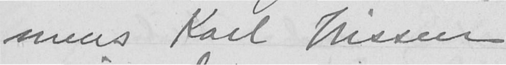mens Karl Nissen
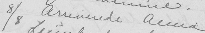8/8 Arriverede Anna
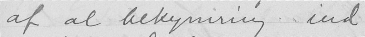af al bekymring ind
